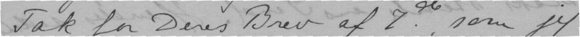Tak for Deres Brev af 7de, som jeg
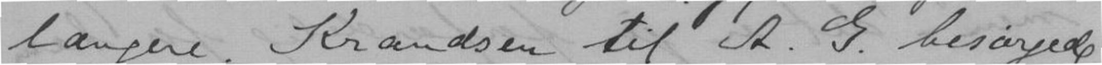længere. Krandsen til A. G. besørgede
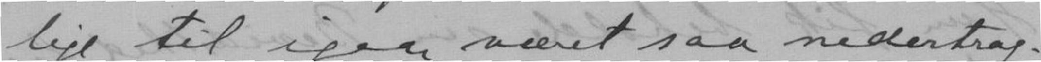lige til igaar været saa nedertrag-
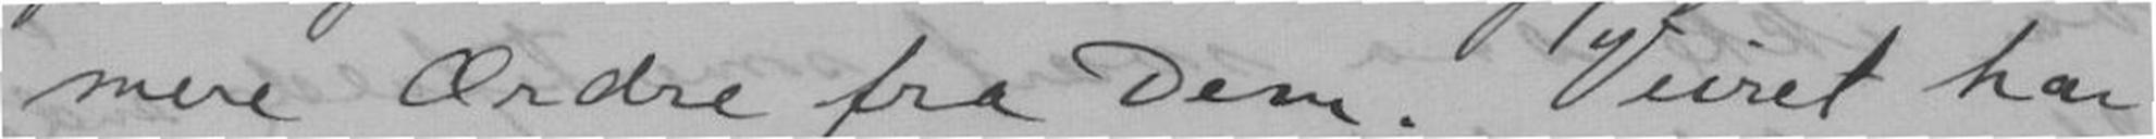mere Ordre fra Dem. Veiret har
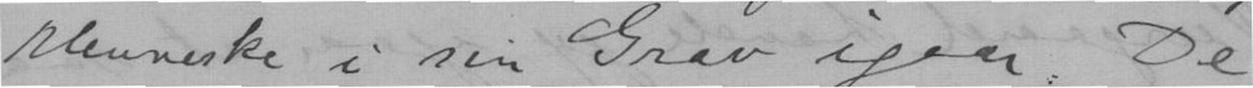Menneske i sin Grav igaar. De
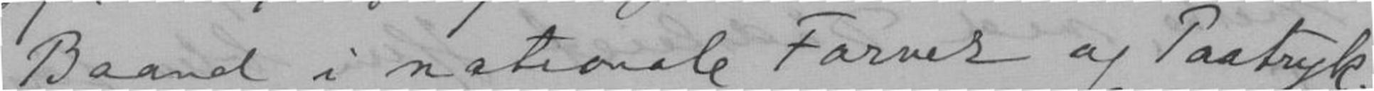Baand i nationale Farver og Paatryk-
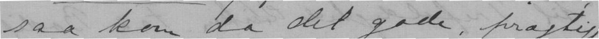saa kom da det gode, prægtige
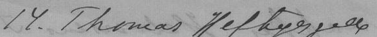14. Thomas Heftyesgade
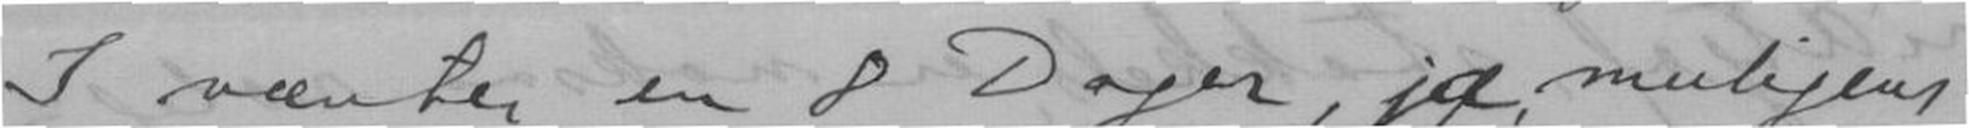I vænter en 8 Dager, ja muligens
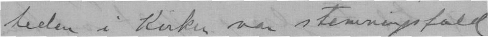heden i Kirken var stemningsfuld
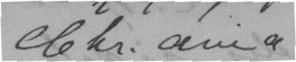Chr.ania
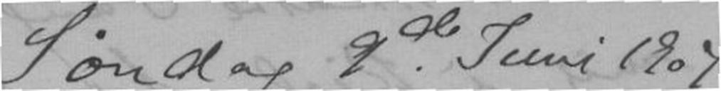Søndag 9de Juni 1907
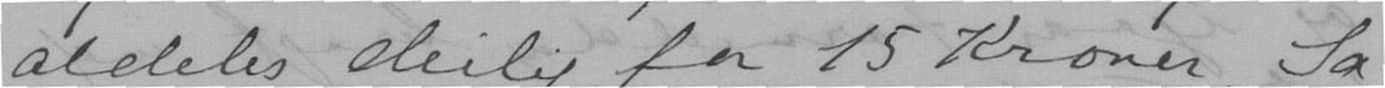aldeles deilig for 15 Kroner. Ja,
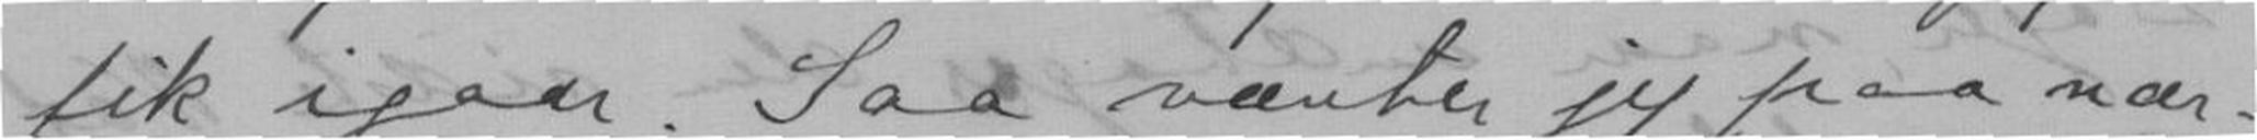fik igaar. Saa vænter jeg paa nær-
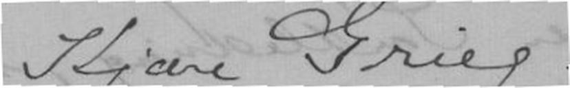Kjære Grieg!
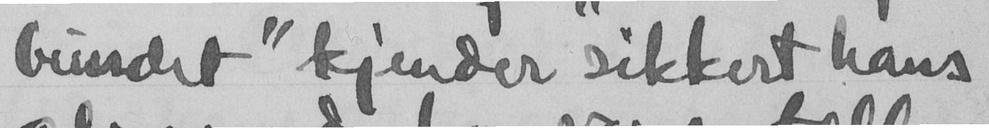bundet" kjender sikkert hans
